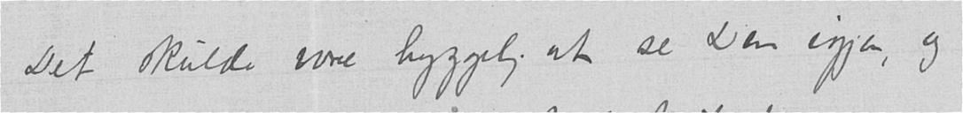Det skulde være hyggelig at se Dem igjen, og
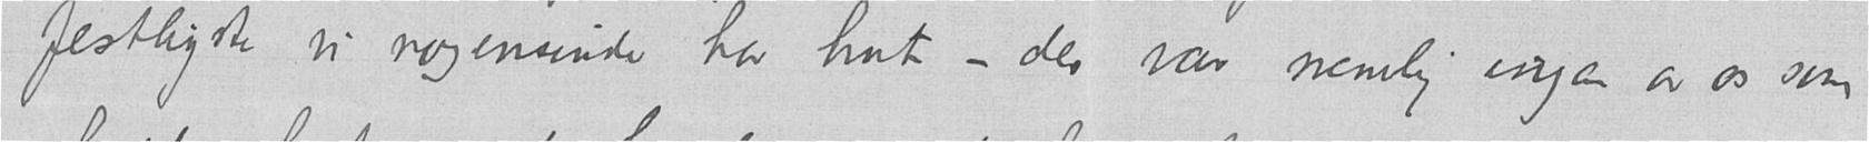festligste vi nogensinde har hat – der var nemlig ingen av os som
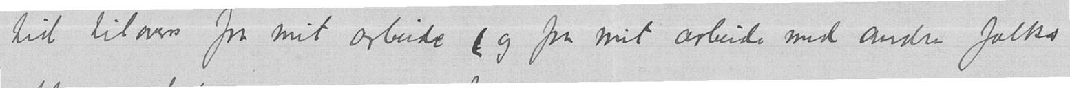tid tilovers fra mit arbeide (og fra mit arbeide med andre folks
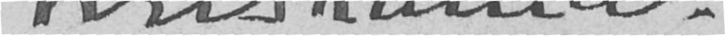Kristiania.
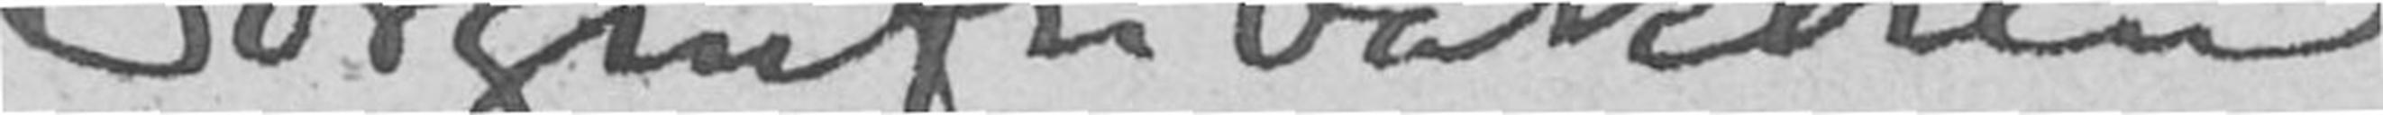Sorgenfribakken
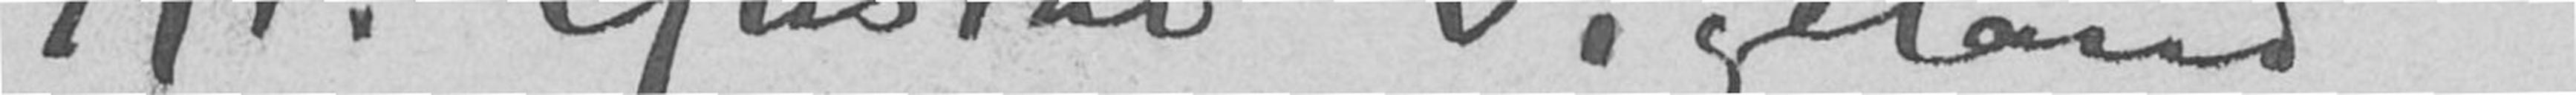Hr. Gustav Vigeland
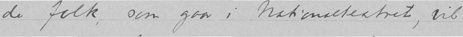de folk, som gaar i Nationalteatret, vil
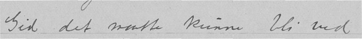Gid det maatte kunne bli ved
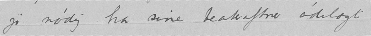jo nødig ha sine teateraftner ødelagt
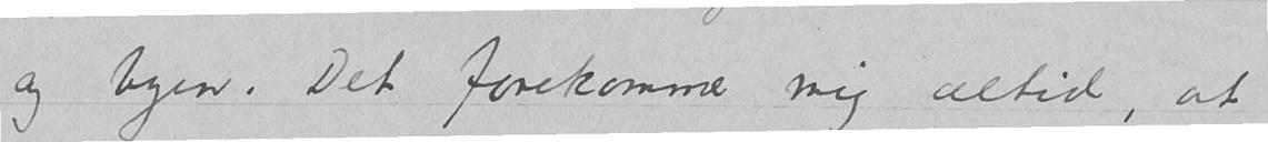og byen. Det forekommer mig altid, at
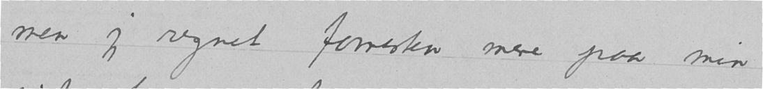men jeg regnet forresten mere paa min
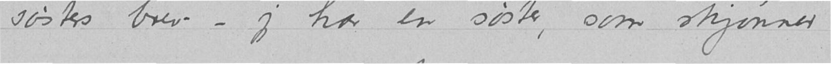søsters brev – jeg har en søster, som skjønner
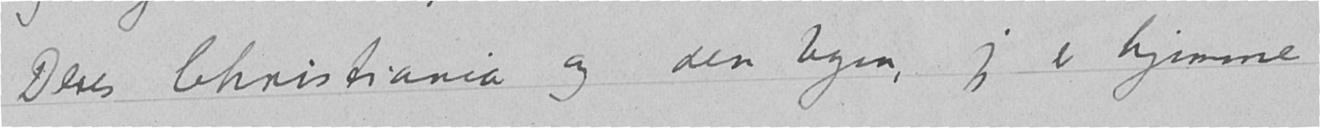Deres Christiania og den byen, jeg er hjemme
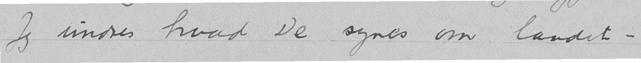Jeg undres hvad De synes om landet –
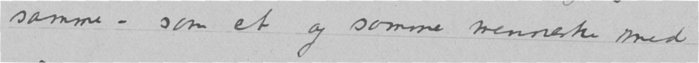samme – som et og samme menneske med
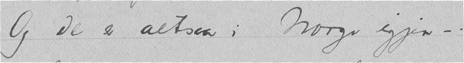Og De er altsaa i Norge igjen - .
